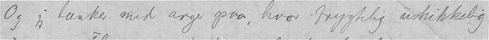Og jeg tænker med anger paa, hvor frygtelig uskikkelig
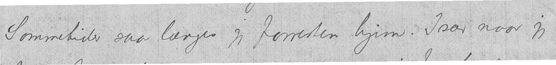Sommetider saa længes jeg forresten hjem. Især naar jeg
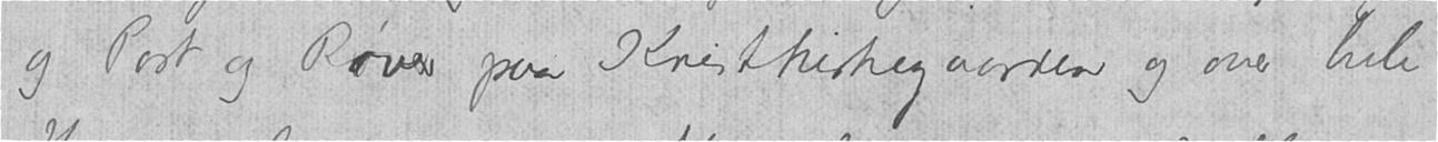og Post og Røver paa Kristkirkegaarden og over hele
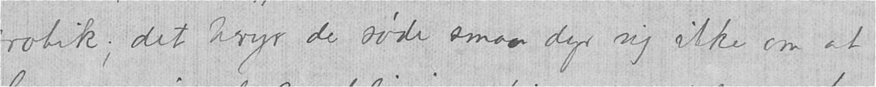erotik; det bryr de søde smaa dyr sig ikke om at
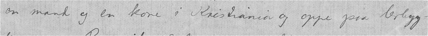en mand og en kone i Kristiania og oppe paa lerbyg-
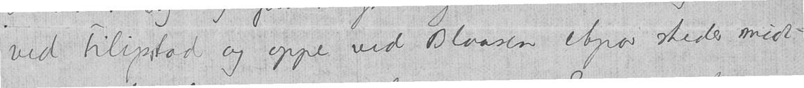ved Filipstad og oppe ved Blaasen etpar steder midt
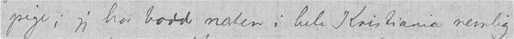pige; jeg har bodd næsten i hele Kristiania nemlig
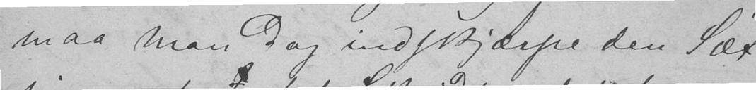maa man dog indskjærpe den Sæt-
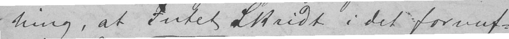ning, at Intet Skridt i det fornuf-
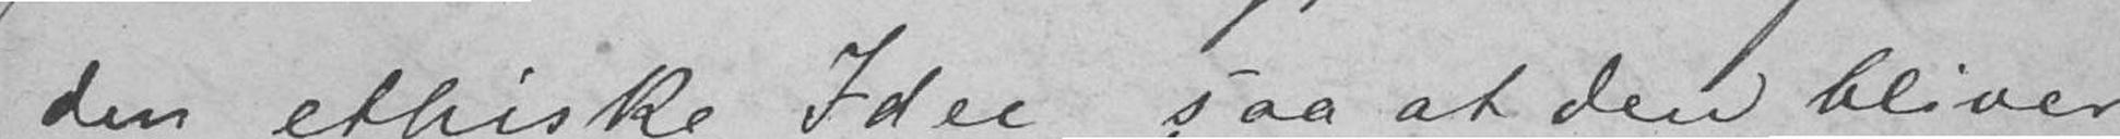den ethiske Idee, saa at den bliver
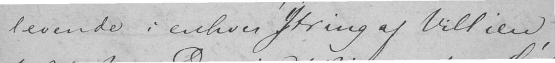levende i enhver Yttring af Villien,
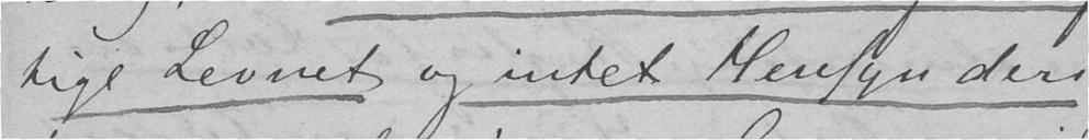tige Levnet og intet Hensyn deri
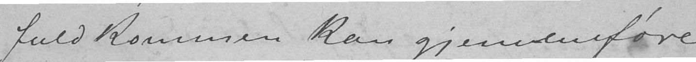fuldkommen kan gjennemføre
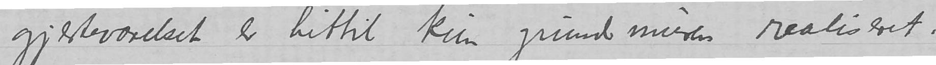gjesteværelset er hittil kun grundmuren realiseret.
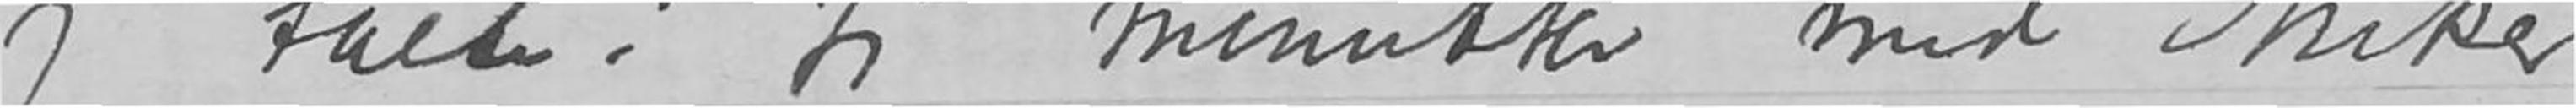jeg talte i ti minutter med Anker
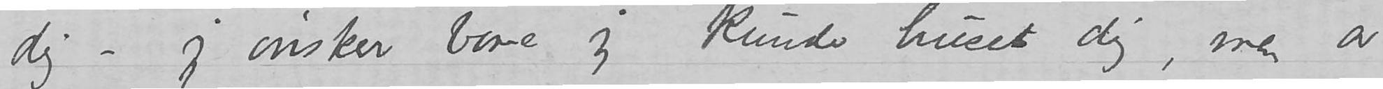dig – jeg ønsker bare jeg kunde huset dig, men av
